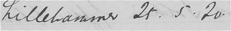Lillehammer 25.5.20.
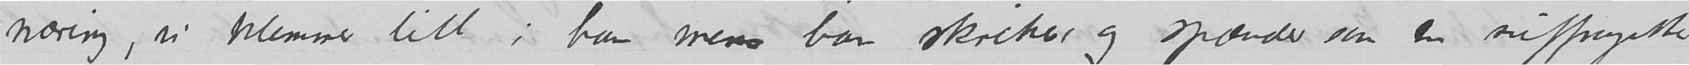næring, vi klemmer litt i ham mens han skriker og spænder som en suffragette
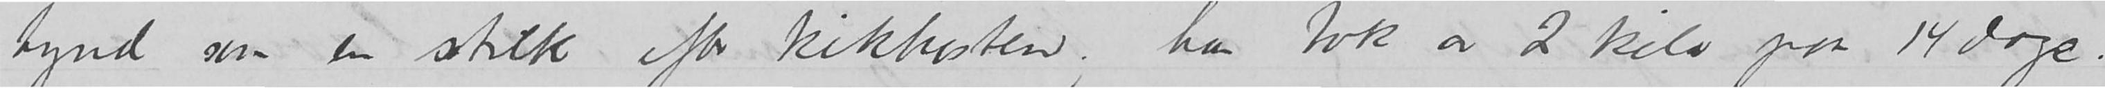tynd som en stilk efter kikhosten; han tok av 2 kilo paa 14 dage.
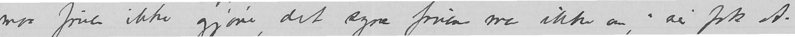maa fruen ikke gjøre, det synes fruen saa ikke om,» sier frk A.
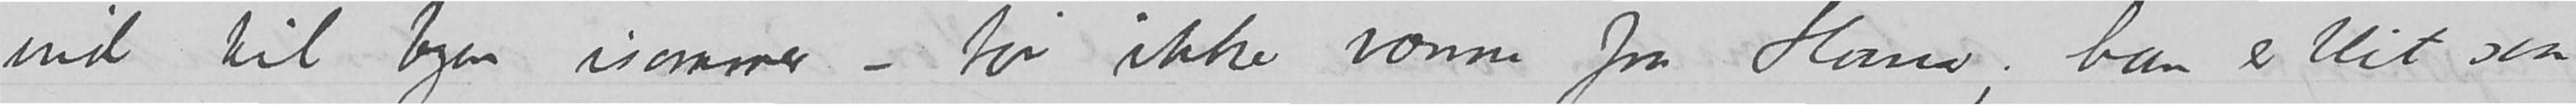ind til byen isommer – tør ikke rømme fra Hans; han er blit saa
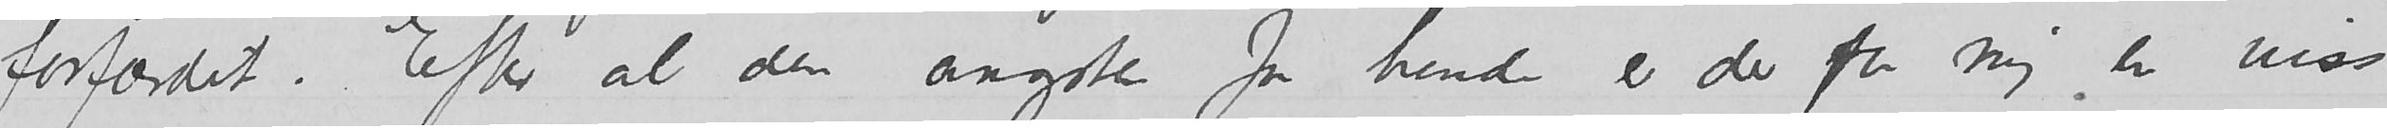forfærdet.  Efter al den angsten for hende er der for mig en viss
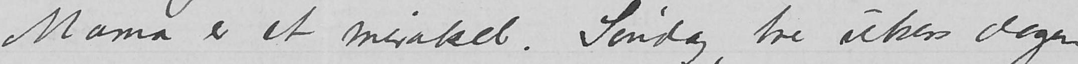Mama er et mirakel. Søndag, tre ukers dagen
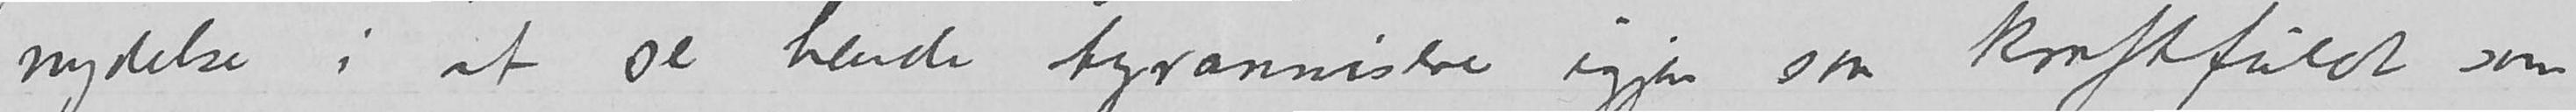nydelse i at se hende tyrannisere igjen saa kraftfuldt som
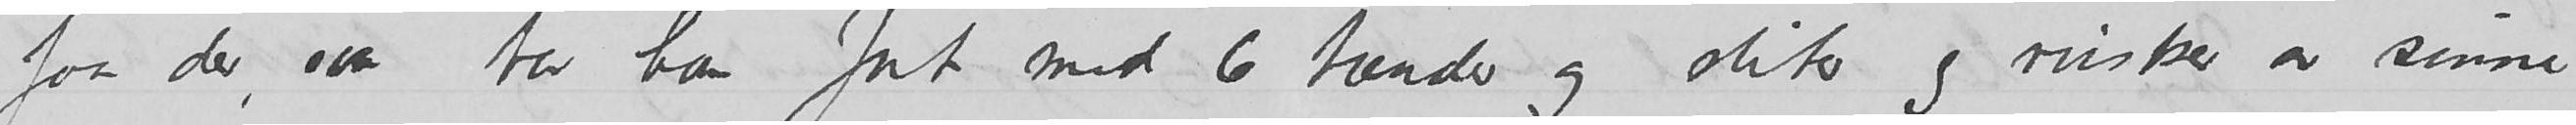faa der, tar han fat med 6 tænder og sliter og rusker av sinne –-.
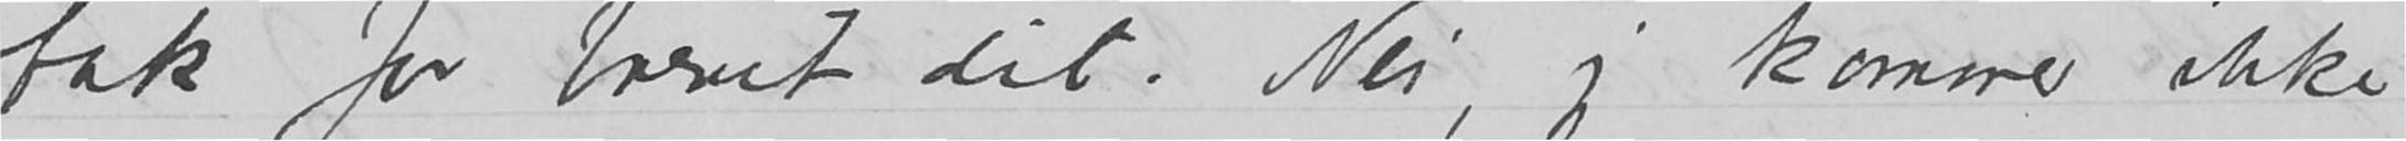tak for brevet dit.  Nei, jeg kommer ikke
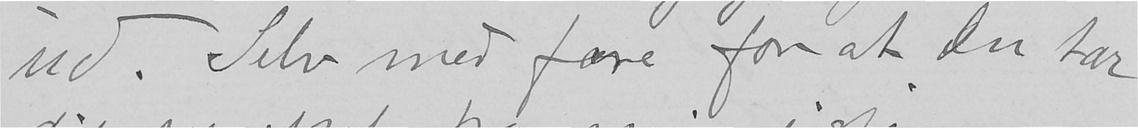ud. Selv med fare for at Du tar
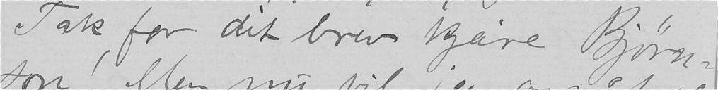Tak for dit brev kjære Bjørn-
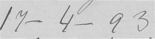17 - 4 - 93
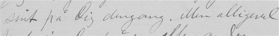sint på Dig dengang. Men alligevel
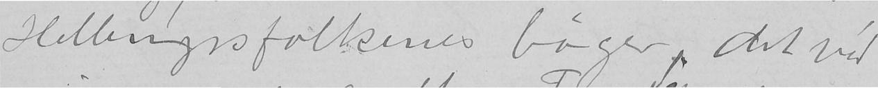Hellemyrsfolkenes bøger, det véd
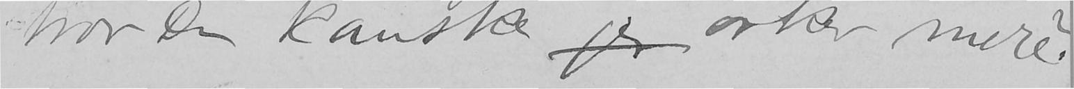tror Du kanske jeg orker mere?
